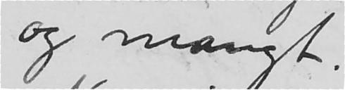og mangt.
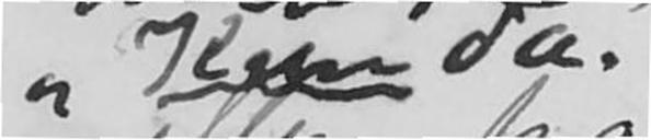Kun da.
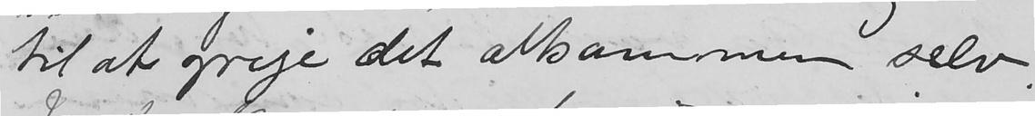til at greje det altsammen selv.
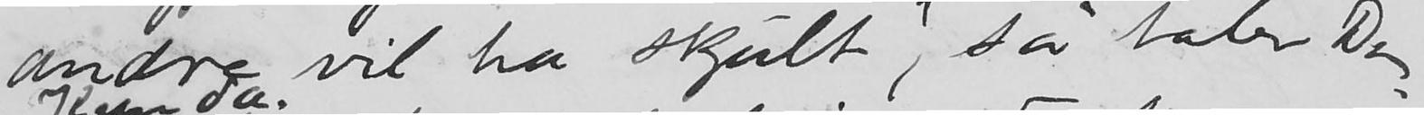andre vil ha skjult, så taler Du.
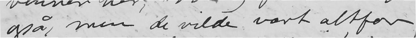også, men de vilde vært altfor
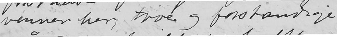venner her, troe og forstandige
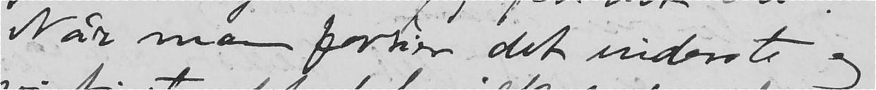Når man fortier det inderste og
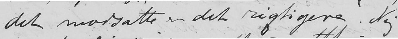det modsatte er det rigtigere. Nej
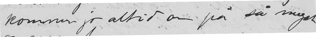kommer jo altid an på så meget
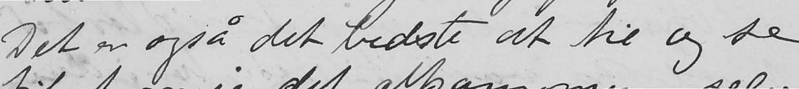Det er også det bedste at til og se
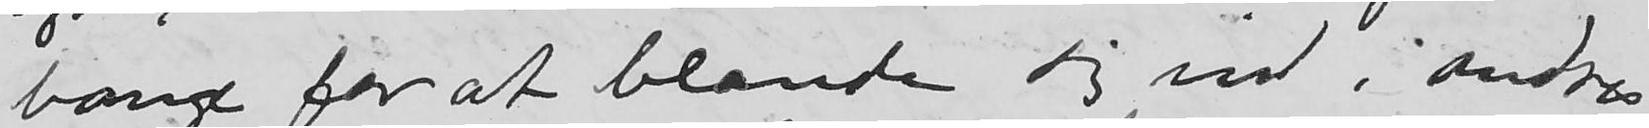bange for at blande sig ind i andres
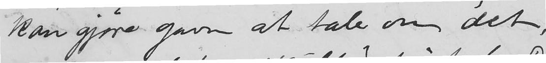kan gjøre gavn at tale om det,
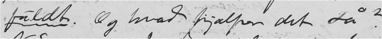fuldt. Og hvad hjælper det så?
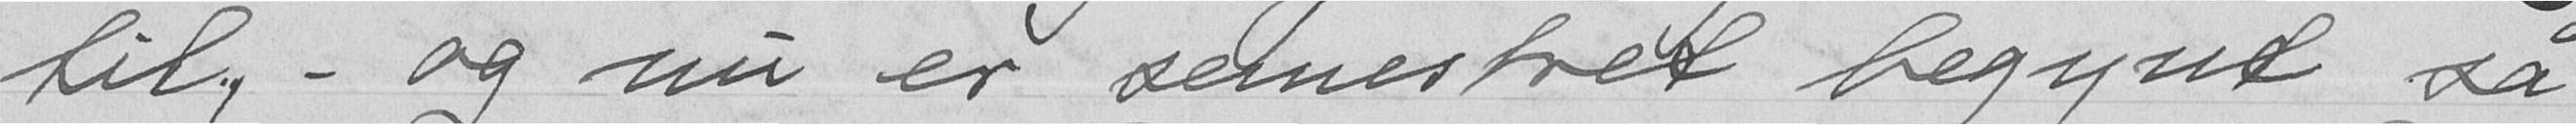til,- og nu er semestret begynt så
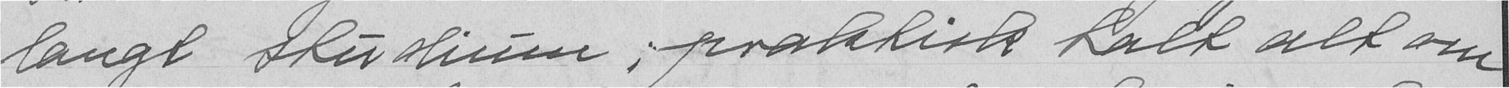langt studium; praktisk talt alt om
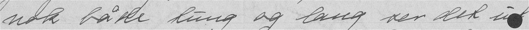nok både tung og lang set det ut
